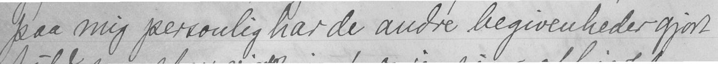paa mig personlig har de andre begivenheder gjort
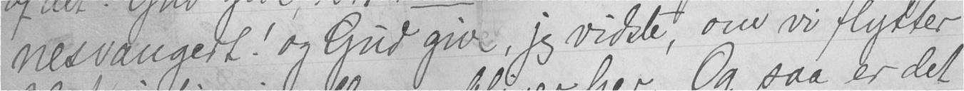nesvangert! og Gud give, jeg vidste, om vi flytter
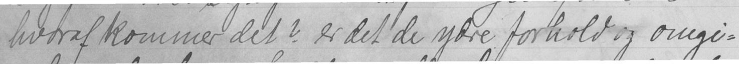hvoraf kommer det? er det de ydre forhold og omgi-
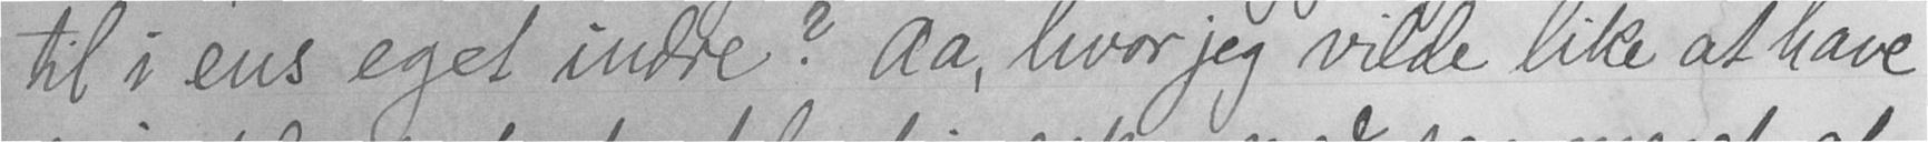til i ens eget indre? Aa, hvor jeg vilde like at have
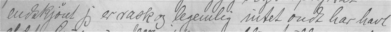endskjønt jeg er rask og legemlig intet ondt har havt
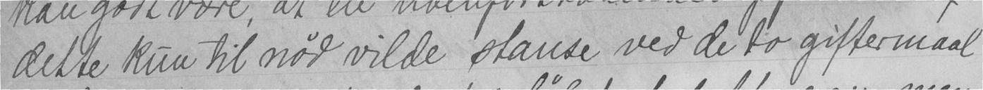dette kun til nød vilde stanse ved de to giftermaal
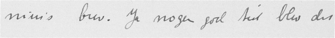nius's brev. Ja nogen god tid blev det
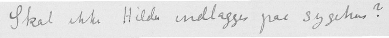Skal ikke Hilde indlægges paa sygehus?
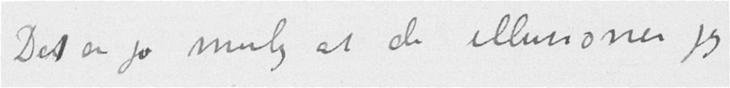Det er jo mulig at de illusioner jeg
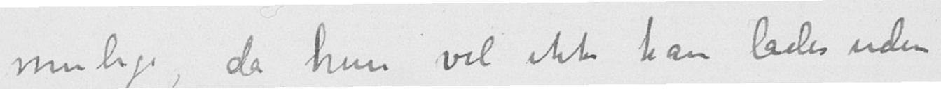mulige, da hun vel ikke kan lades uden
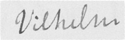Vilhelm
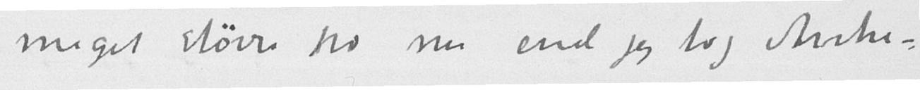meget større ro nu end jeg tog Arrhe-
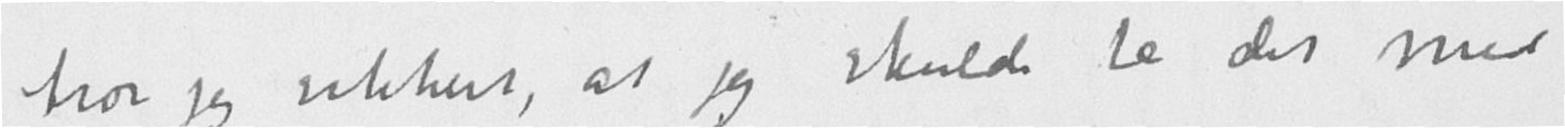tror jeg sikkert, at jeg skulde ta det med
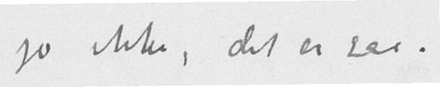jo ikke, det er saa.
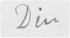Din
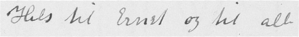Hils til Ernst og til alle
